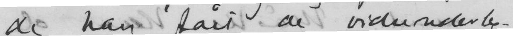de han fået de vidunderlig-
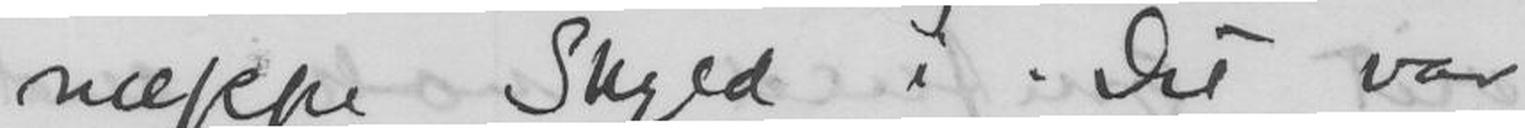næppe Skyld i. Det var
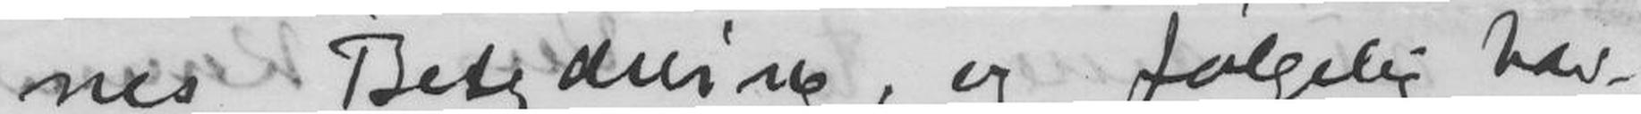nes Betydning, og følgelig hav-
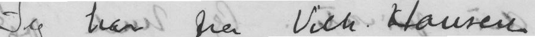Jeg har fra Vilh. Hansen
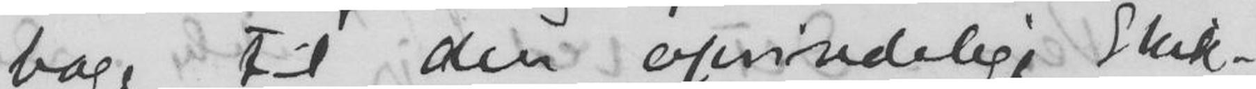bage til den oprindelige Skik-
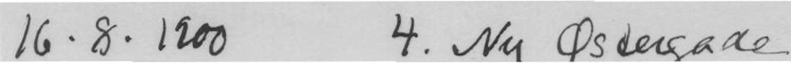16.8.19004 Ny Østergade
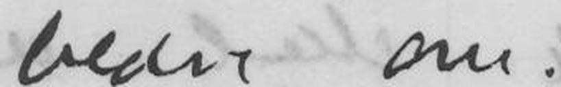bedre om.
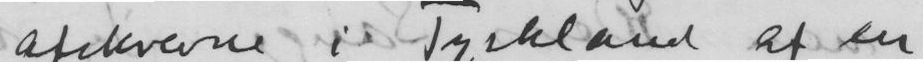afskrevne i Tyskland af en
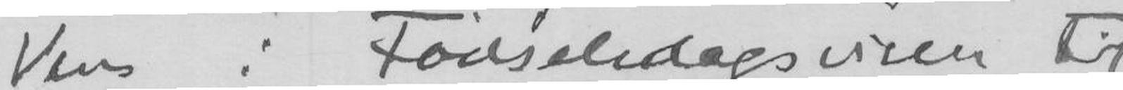Vers i Fødselsdagsvisen til
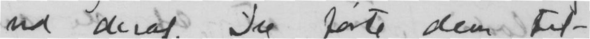ud deraf. Jeg førte dem til-
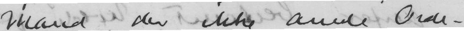mand, der ikke anede Orde-
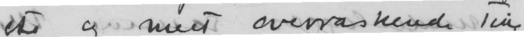ste og mest overraskende Ting
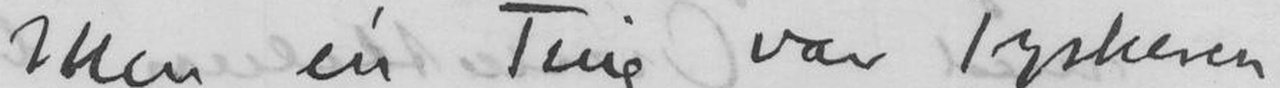Men èn Ting var Tyskeren
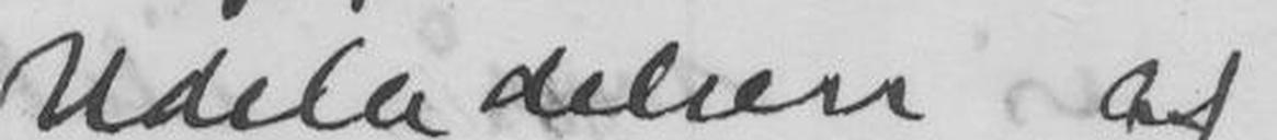Udeladelsen af
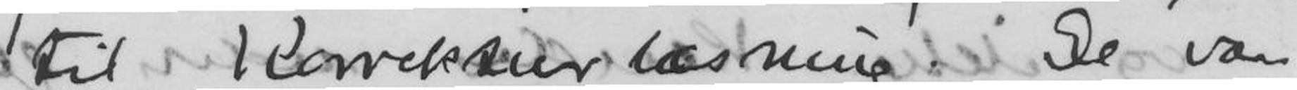til Korrekturlæsning. De var
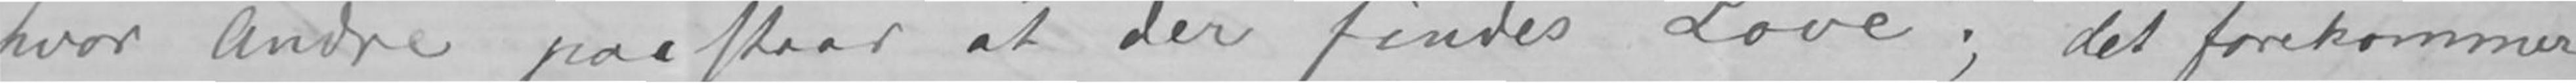hvor Andre paastaar at der findes Love; det forekommer
